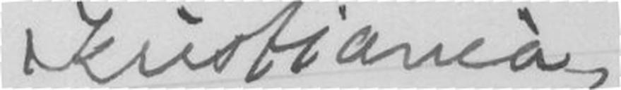Kristiania
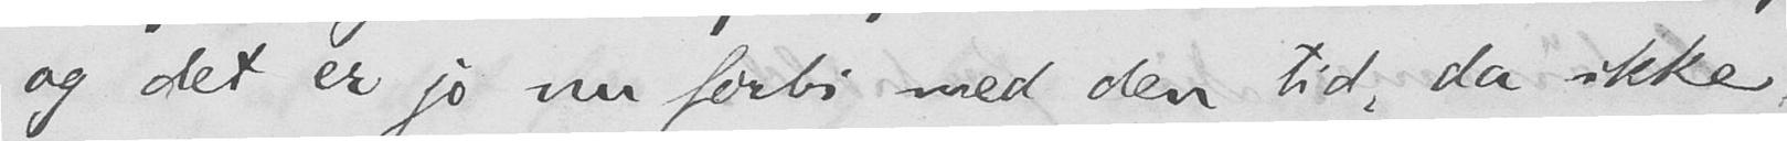og det er jo nu forbi med den tid, da ikke
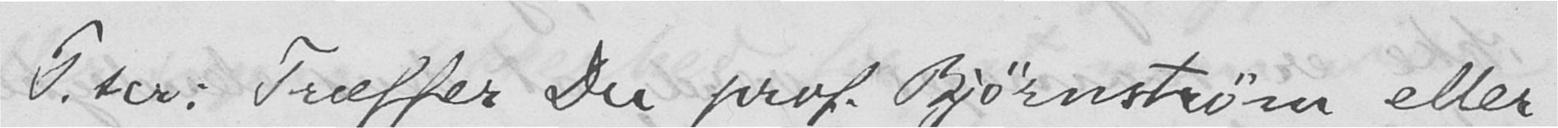P. scr: Træffer Du prof. Bjørnstrøm eller
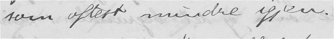som oftest mindre igjen.
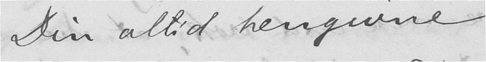Din altid hengivne
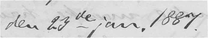den 23de jan. 1887.
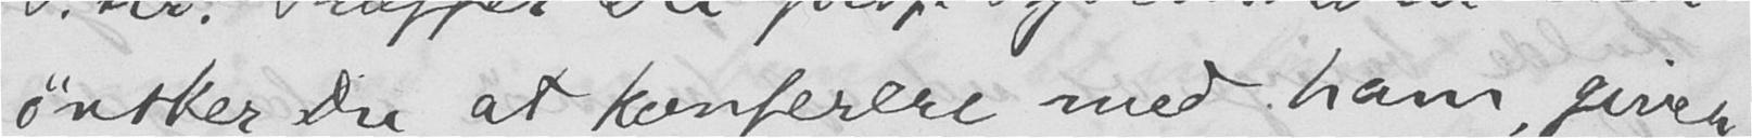ønsker Du at konferere med ham, giver
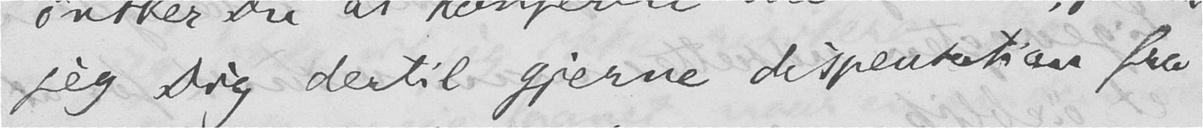jeg Dig dertil gjerne dispensation fra
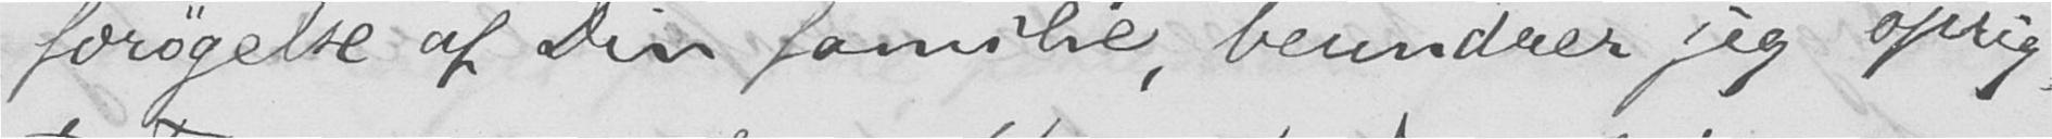forøgelse af Din familie, beundrer jeg oprig-
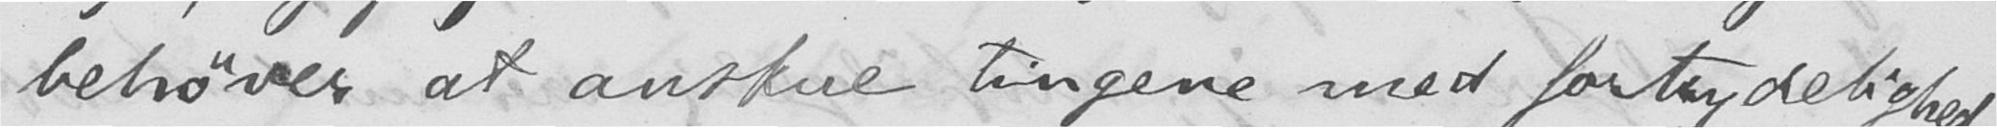behøver at anskue tingene med fortydelighed.
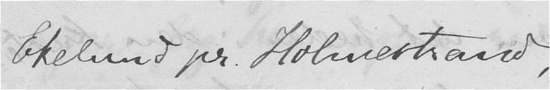Ekelund pr. Holmestrand,
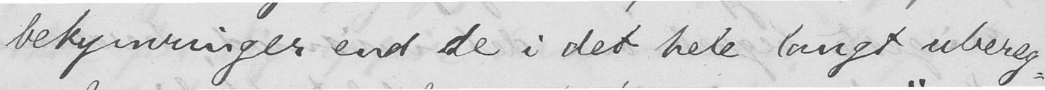bekymringer end de i det hele langt ubereg-
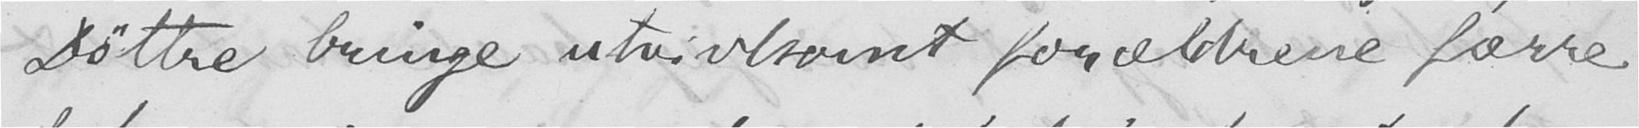Døttre bringe utvivlsomt forældrene færre
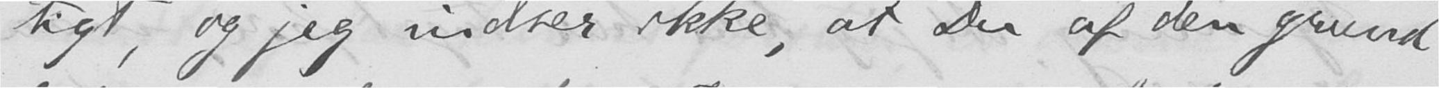tigt, og jeg indser ikke, at Du af den grund
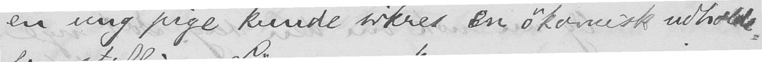en ung pige kunde sikres en økomisk udholde-
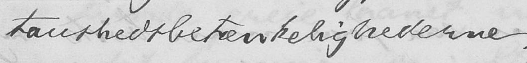taushedsbetænkelighederne.
